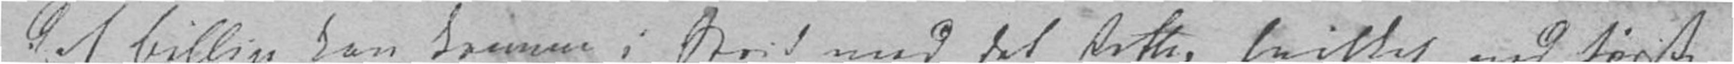det Billige kan komme i Strid med det Rette, hvilket ved første
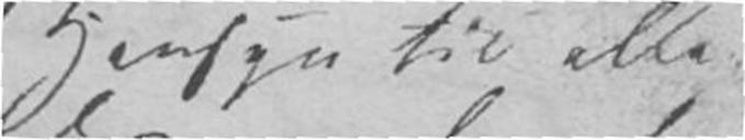Hensyn til alle
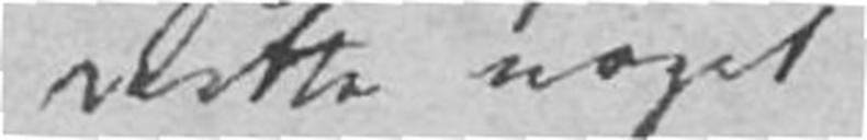Rette noget
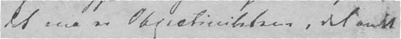det ene er Objectivitetens, det andet
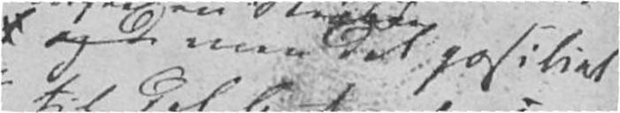og de men det positivt
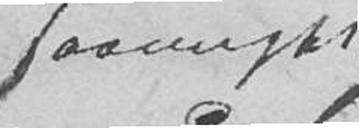saameget
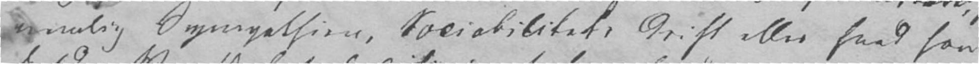nemlig Sympathien, Sociabilitet, Drift eller hvad han
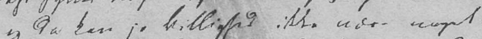og da kan jo Billighed ikke være noget
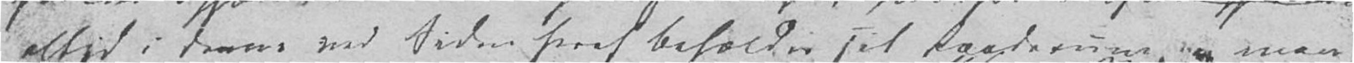altid i denne ved Siden heraf beholder sit Raaderum, og men
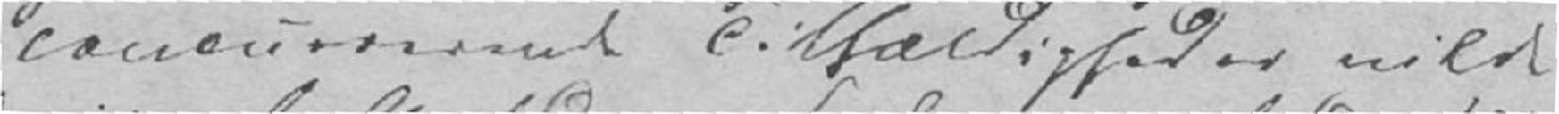concurrerende Tilfældigheder vilde
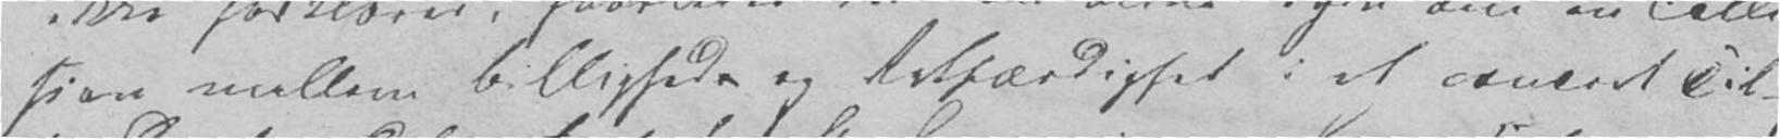sion mellem Billighede og Retfærdighed i et concret Til-
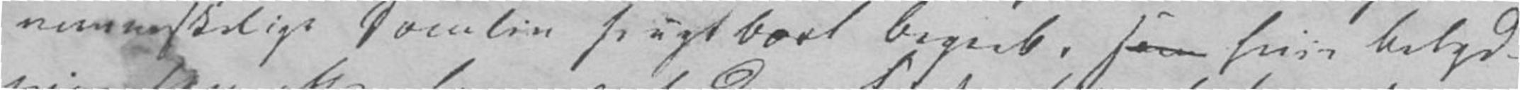menneskelige Samliv frugtbart Begreb, som hvis Betyd-
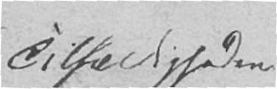Tilfældigheden
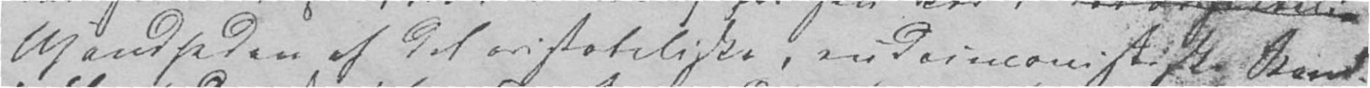Usandheden af det aristoteliske, eudaimonistiske Stand-
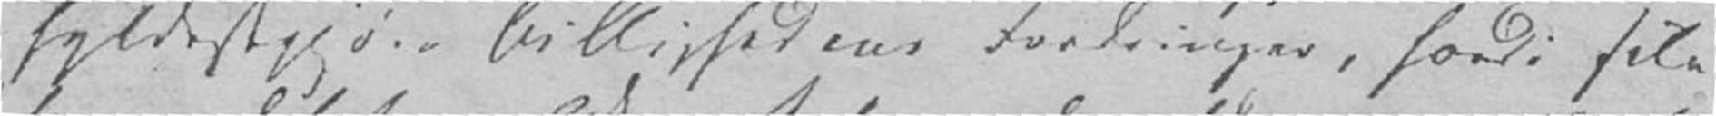fyldestgjøre Billighedens Fordringer, fordi selv
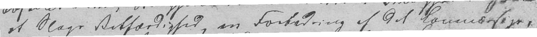et Slags Retfærdighed, en Forbedring af det Lovmæssige,
